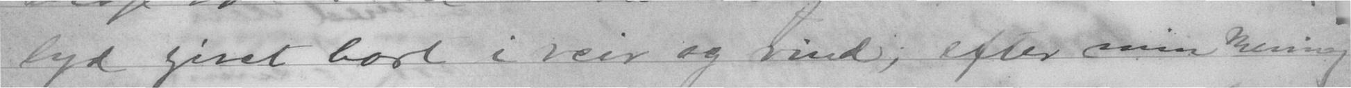lyd givet bort i veir og vind, efter min meining
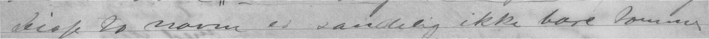Disse to navn er sanderlig ikke bare tommes
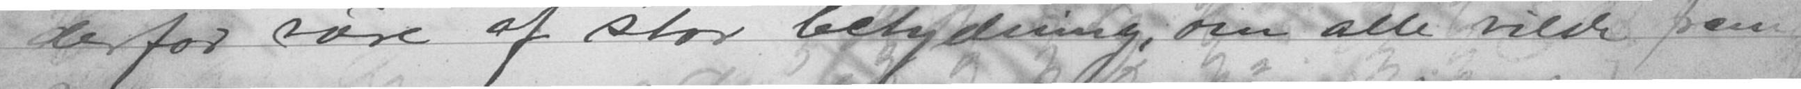derfor være af stor betydning om alle vilde frem-
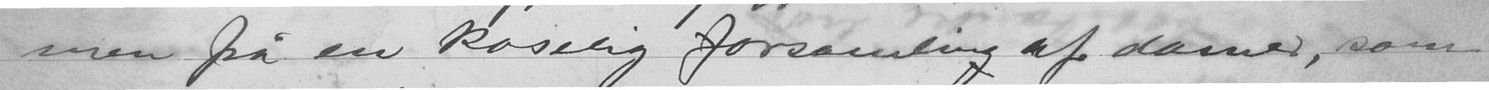men på en koselig forening af damer, som
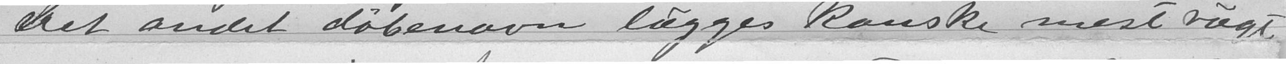det andet døbenavn læggges kanske mest vægt
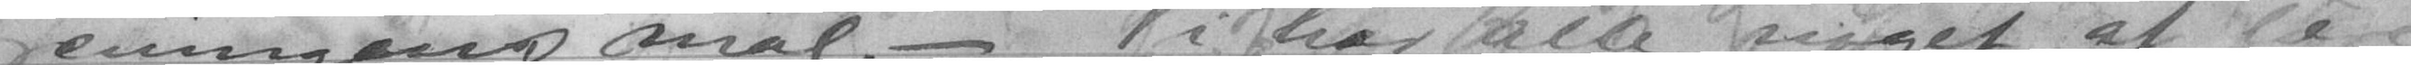foreningens mål. - Vi har alle meget at lære
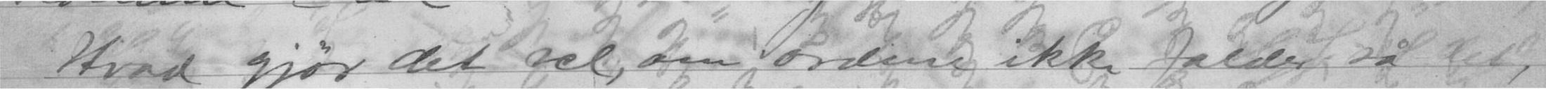Hvad gjør det vel, om ordene ikke falder så
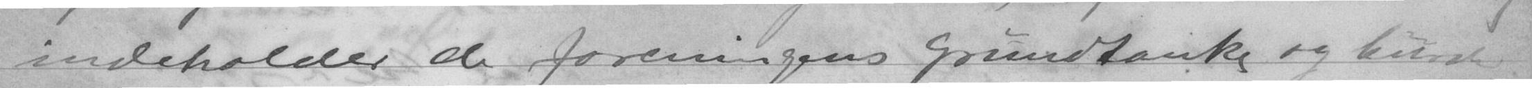indeholder de foreningens grundtanker og be
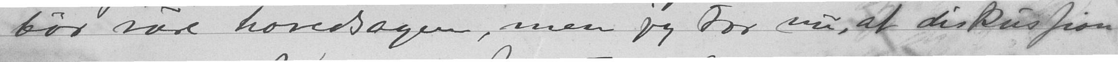bør være hovedsagen, men jeg tror nu, at diskution
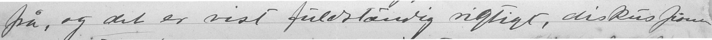på, og det er vist fuldstendig rigtigt, diskusitionen
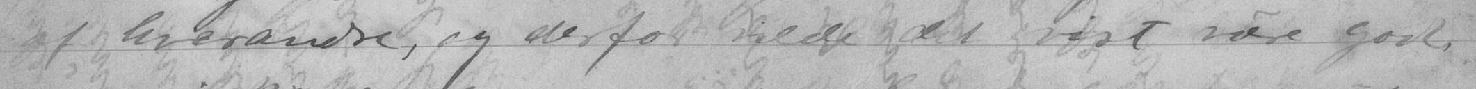af hverandre, og derfor vilde det vist være godt,
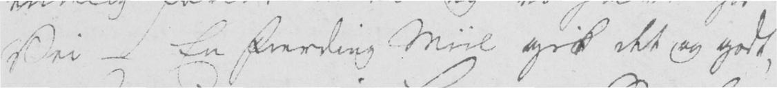Vei - En fierding Miil gik det og godt,
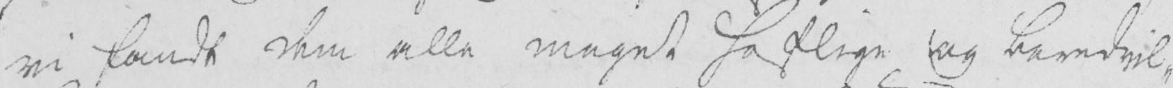vi fandt dem alle meget høflige og beredvil-
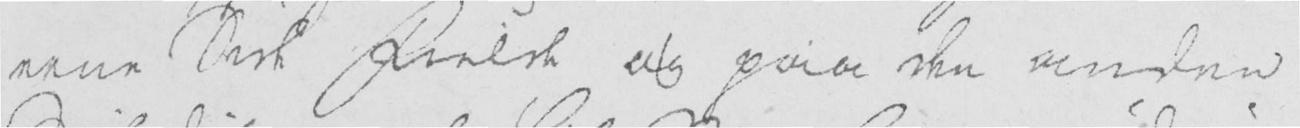eene Side Fielde og paa den anden
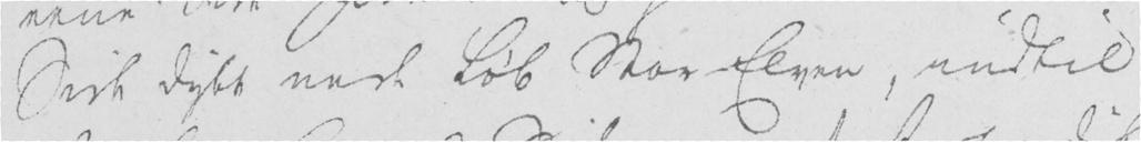Side dybt nede løb Stor-Elven, indtil
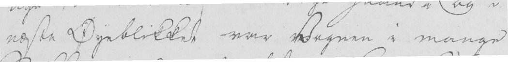næste Øyeblikket var Vognen i mange
